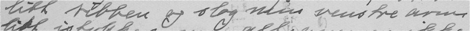litt ribben og slog min venstre arm
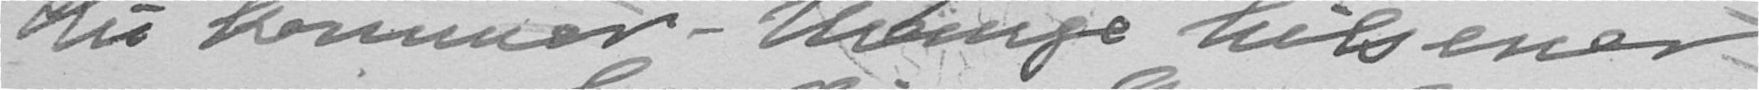du kommer - Mange hilsener
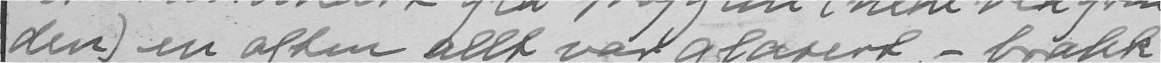den) en aften allt var glasert - brakk
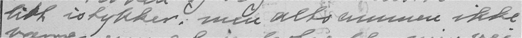litt istykker, men altsammen ikke
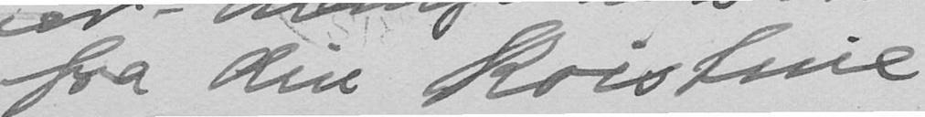fra din Kristine
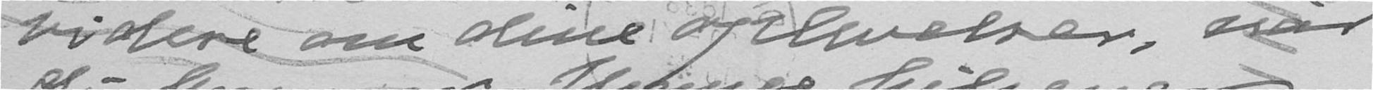videre om dine oplevelser, når
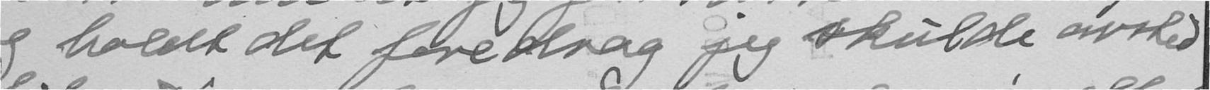og holdt det foredrag jeg skulde avsted
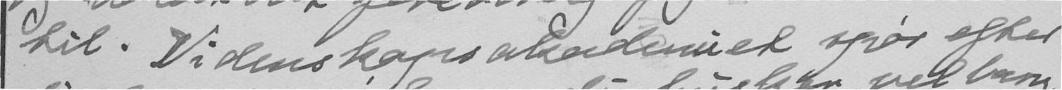til. Videnskapsakademiet spør efter
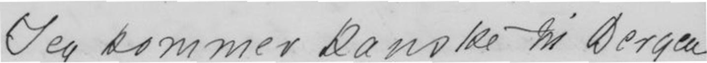Jeg kommer kanske til Bergen
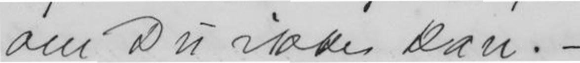om Du ikke kan.-
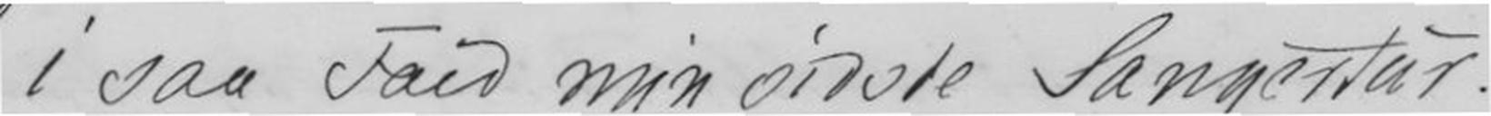i saa Fald min sidste Sangertur.
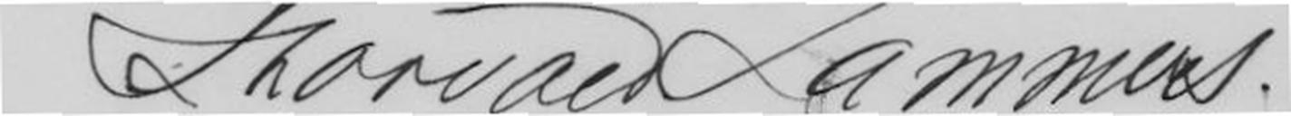Thorvald Lammers
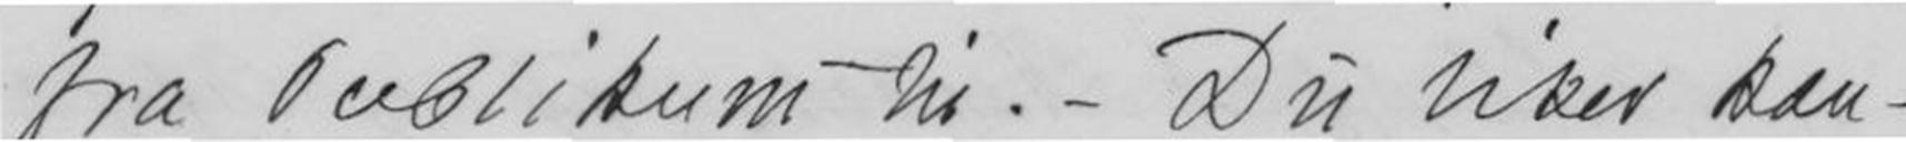fra publikum til.- Du liker kan-
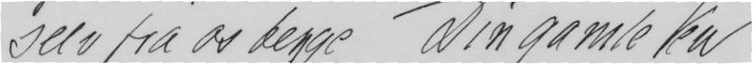selv fra os begge Din gamle Ven
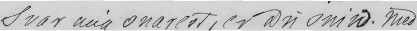Svar mig snarest, er Du snild. Med
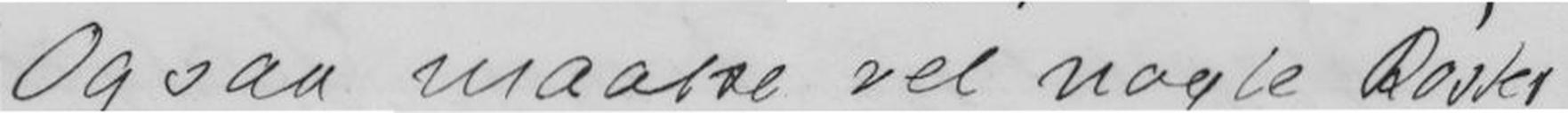Og saa maatse vel nogle Røster
